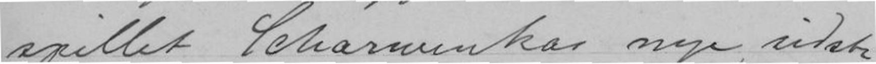spillet Scharwenkas nye sidste
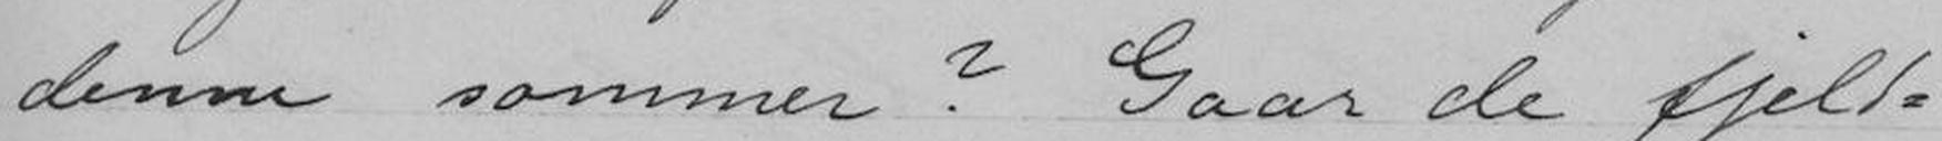denne sommer? Gaar de fjeld-
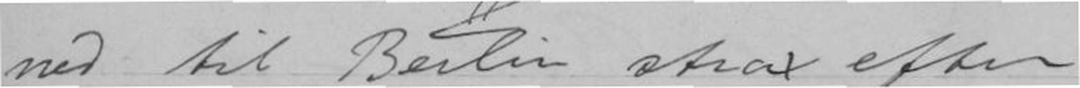ned til Berlin strax efter
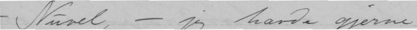- Nuvel, - jeg havde gjerne
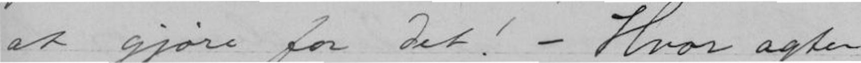at gjøre for det! - Hvor agter
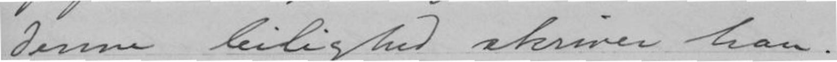denne leilighed skriver han.
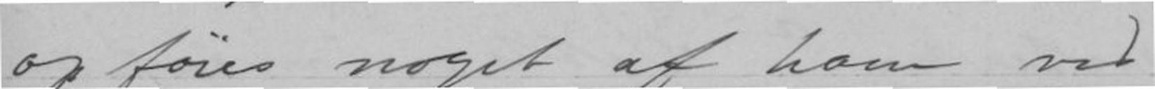opføres noget af ham ved
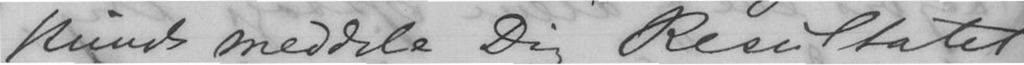Minds meddele Dig Resultatet
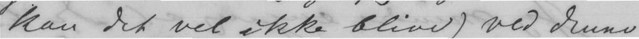kan det vel ikke blive) ved denne
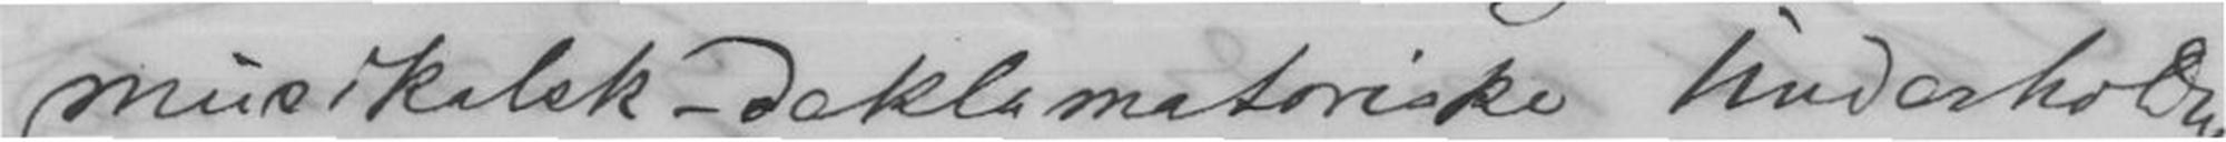musikalske-daklamatariske Underholdning
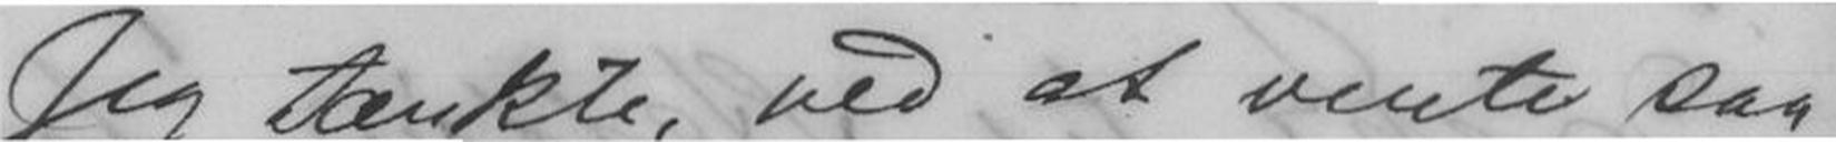Jeg tænkte ved at vente saa
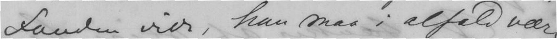Fanden vide, han maa i
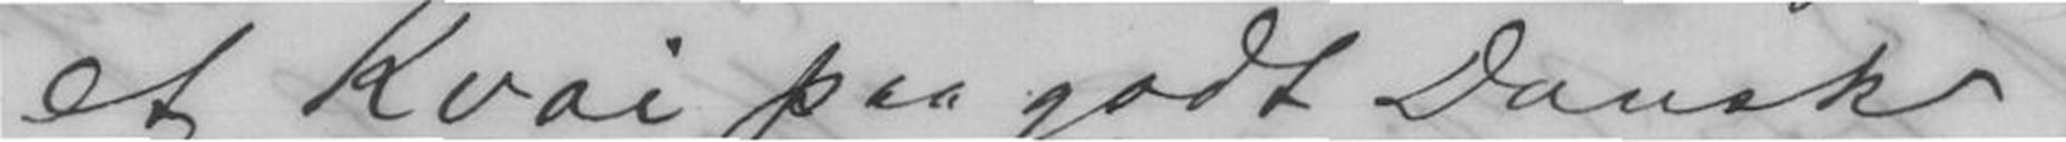altfald være et Kvai paa godt Dansk.
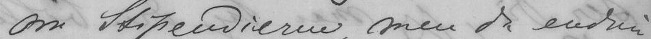om Stipendierne, men da endnu
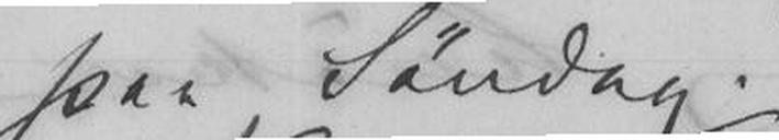paa Søndag.
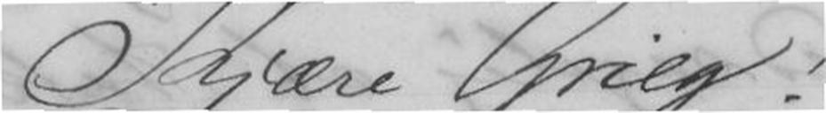Kjære Grieg!
